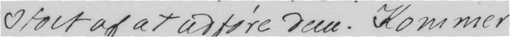stolt af at udføre dem. Kommer
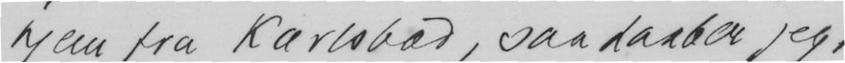hjem fra Karlsbad, saa haaber jeg,
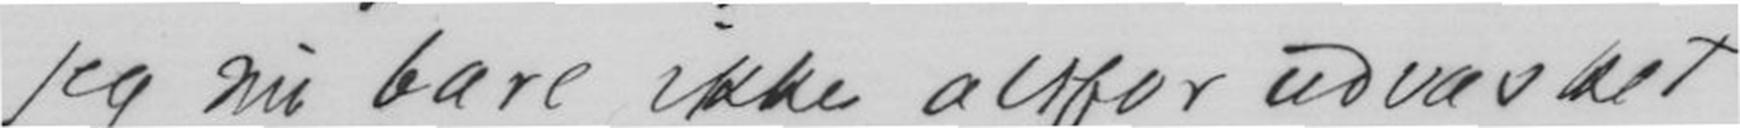jeg nu bare ikke alfor udvasket
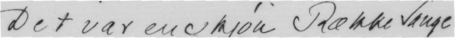Det var en skjøn Række Sange
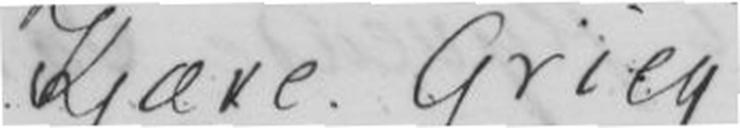Kjære Grieg
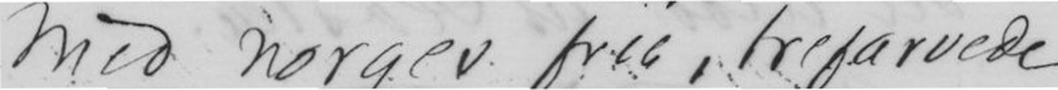Med Norge fra, trefarvede
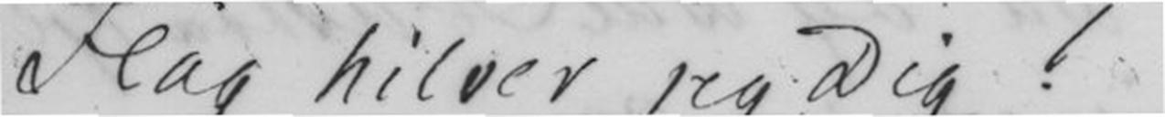Flag hilser jeg Dig!
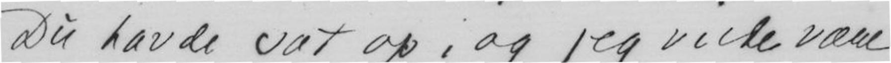Du havde sat op, og jeg vilde være
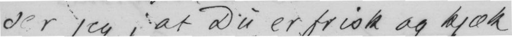ser jeg, at Du er frisk og kjæk,
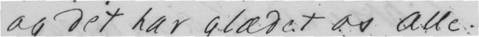og det har glædet os alle.
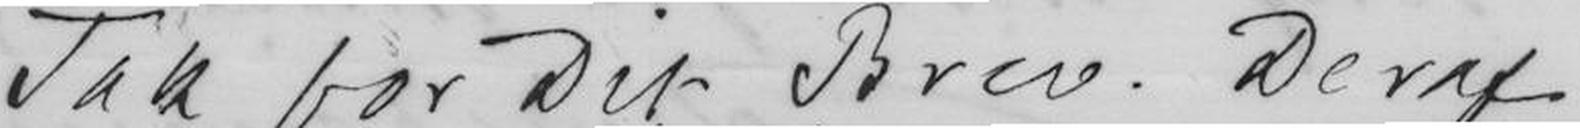Tak for Dit Brev. Deraf
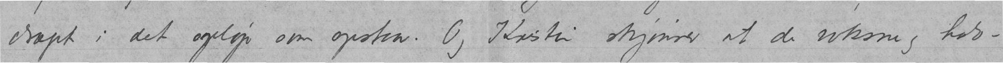dræpt i det opløp som opstaar. Og Kristin skjønner at de voksne og halv-
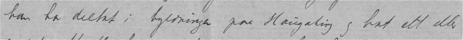han har deltat i hyldningen paa Haugating og hat ett eller
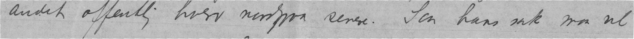andet offentlig hverv nordpaa senere. Saa hans sak maa vel
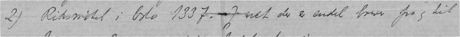2) Riksmøtet i Oslo 1337.  Jeg vet der er en del brever fra og til
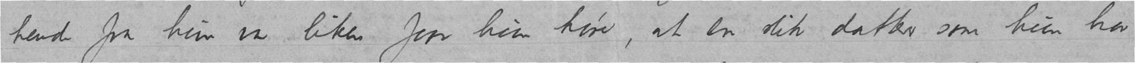hende fra hun var liten faar hun høre, at en slik datter som hun har
